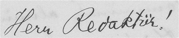Herr Redaktør!
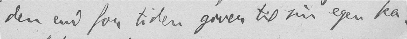den end for tiden giver til sin egen ka-
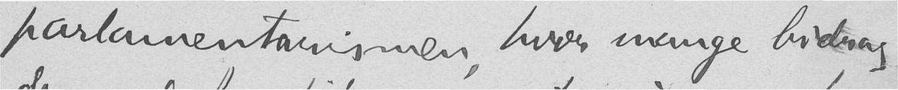parlamentarismen, hvor mange bidrag
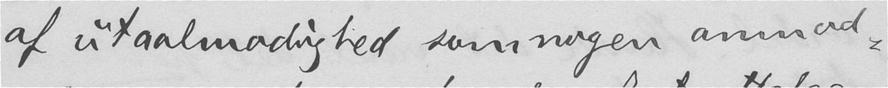af utaalmodighed som nogen anmod-
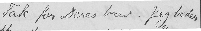Tak for Deres brev. Jeg beder
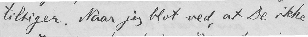tilsiger. Naar jeg blot ved, at De ikke
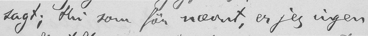sagt; thi som før nævnt, er jeg ingen
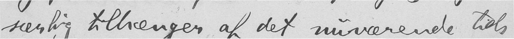særlig tilhænger af det nuværende tids-
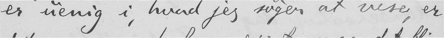er uenig i, hvad jeg søger at vise, er
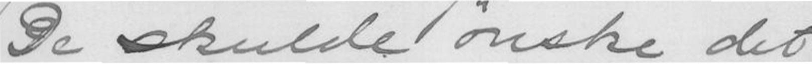De skulde ønske det
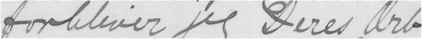forbliver jeg Deres Ærb.
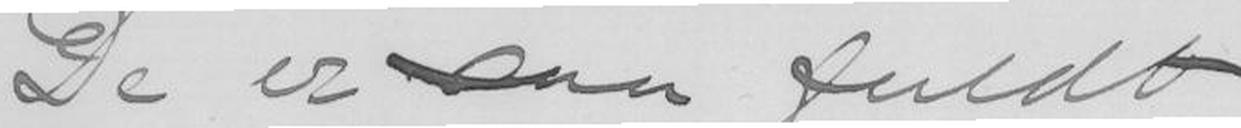De er saa fuldt
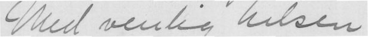Med venlig hilsen
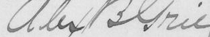Alex B Grieg.
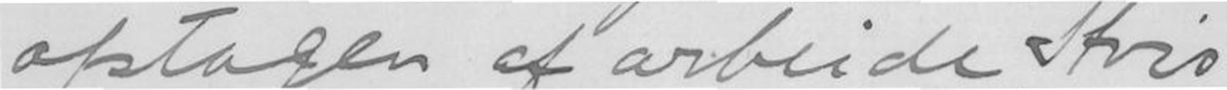optagen af arbeide. Hvis
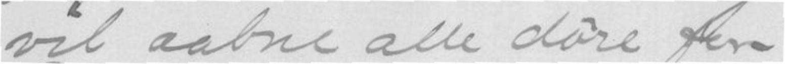vil aabne alle døre for
